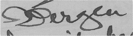Bergen
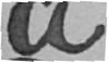a
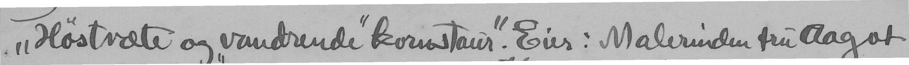"Høstvæte og "vandrende" kornstaur". Eier: Malerinden fru Aagot
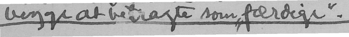bigge at betragte som "færdige".
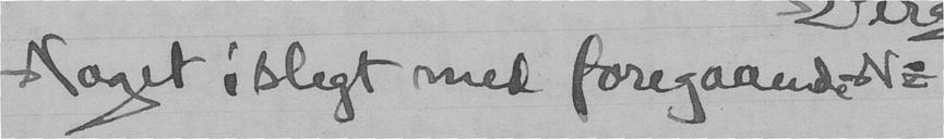Noget i slegt med foregaaende No.
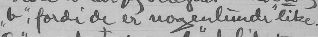"b", fordi de er nogenlunde like.
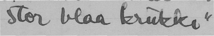stor blaa krukke"
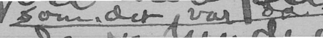som det var da.
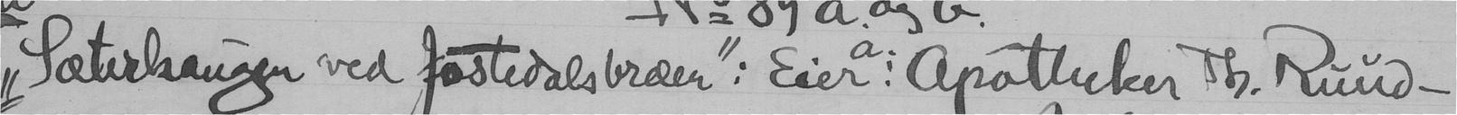"Sæterhaugen ved Jøstedalsbræen": Eier: Apotheker Th. Ruud -
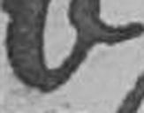b
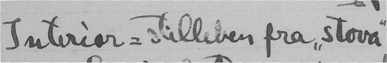"Interior - stilleben fra "stova"
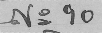No 90
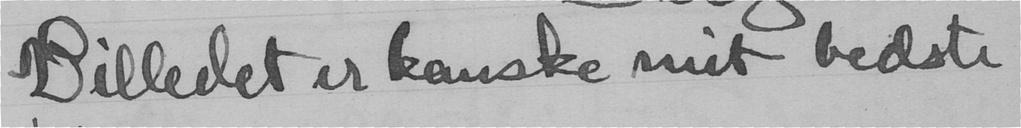Billedet er kanske mit bedste
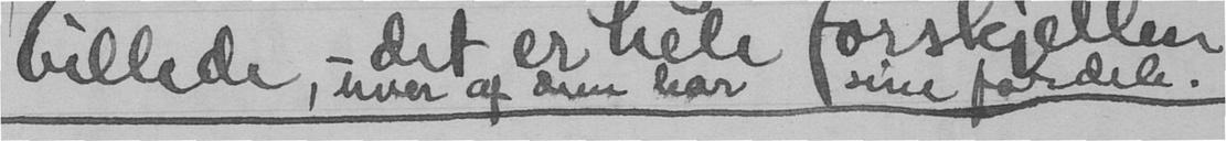billede, hver af dem har sine fordele.
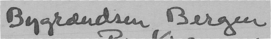Bygrændsen Bergen
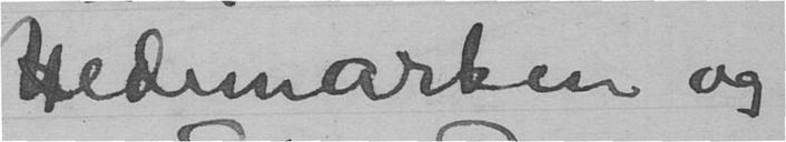Hedemarken og
